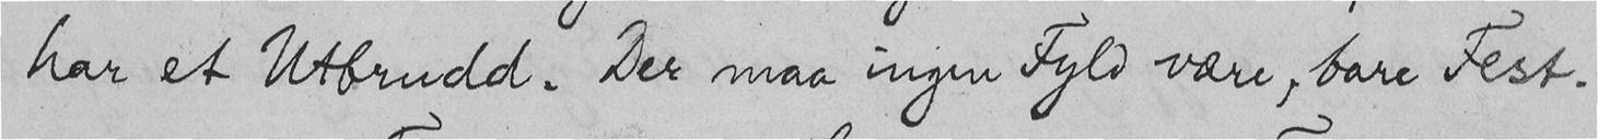har et Utbrudd. Der maa ingen Fyld være, bare Fest.
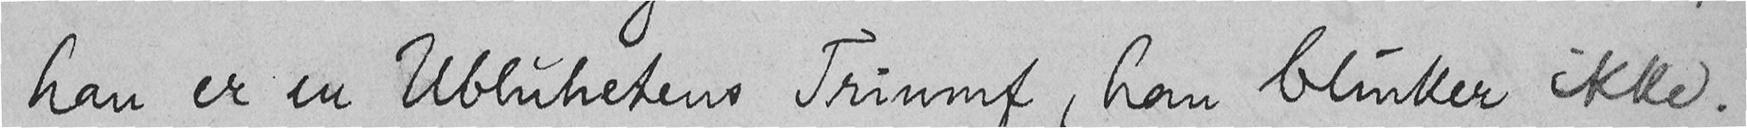han er en Ubluhetens Triumf, han blinker ikke.
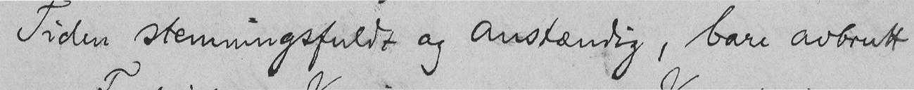Tiden stemningsfuldt og Anstændig, bare avbrutt
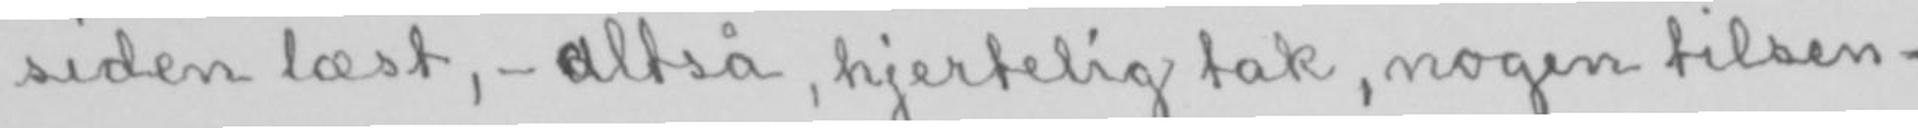siden læst,- altså, hjertelig tak, nogen tilsen-
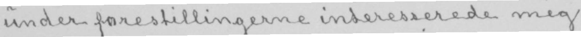under forestillingerne interesserede mig
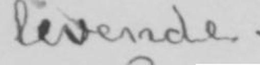levende.
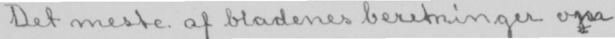Det meste af bladenes beretninger opm
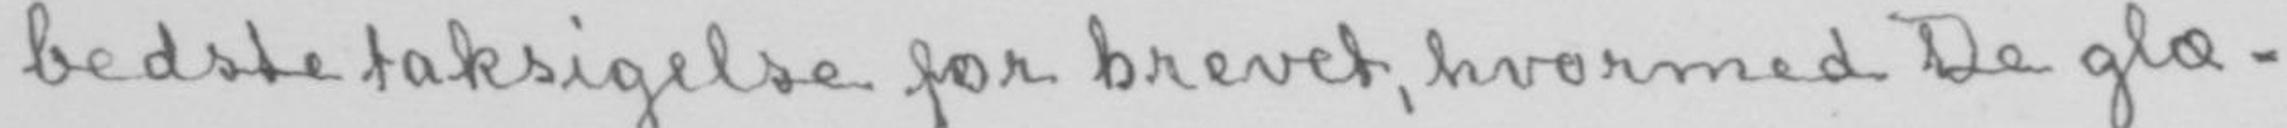beste taksigelse for brevet, hvormed De glæ-
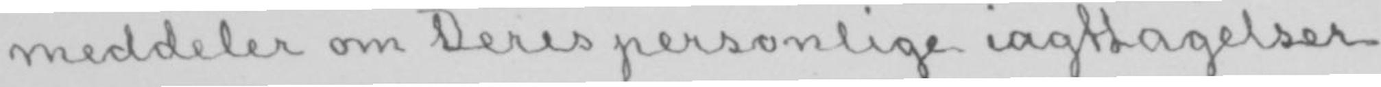meddeler om Deres personlige iagttagelser
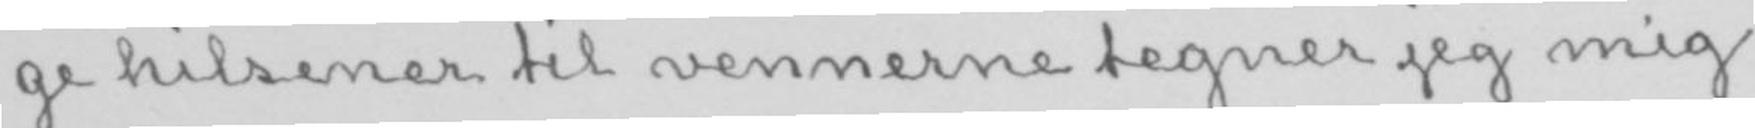ge hilsener til vennerne tegner jeg mig
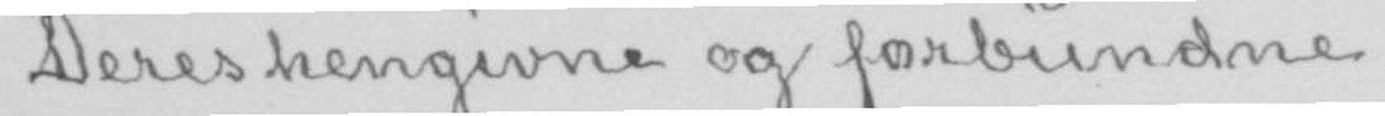Deres hengivne og forbundne
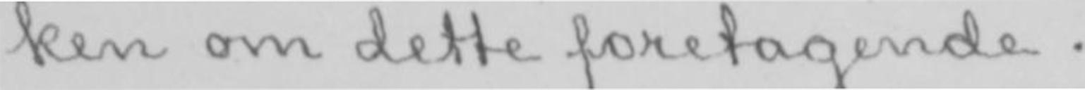ken om dette foretagende.
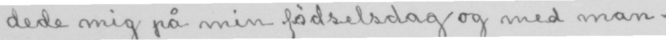dede mig på min fødselsdag og med man-
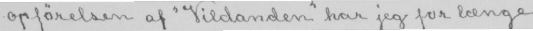opførelsen af «Vildanden» har jeg for længe
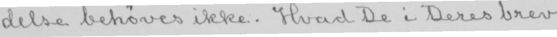delse behøves ikke. Hvad De i Deres brev
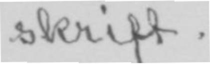skrift.
